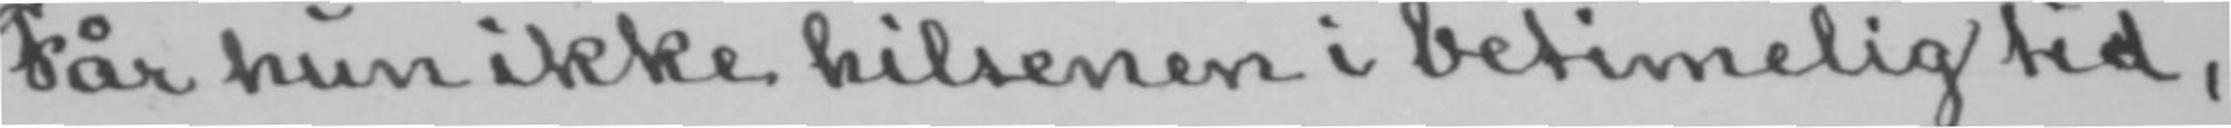Får hun ikke hilsenen i betimelig tid,
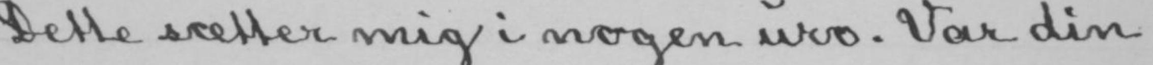Dette sætter mig i nogen uro. Var din
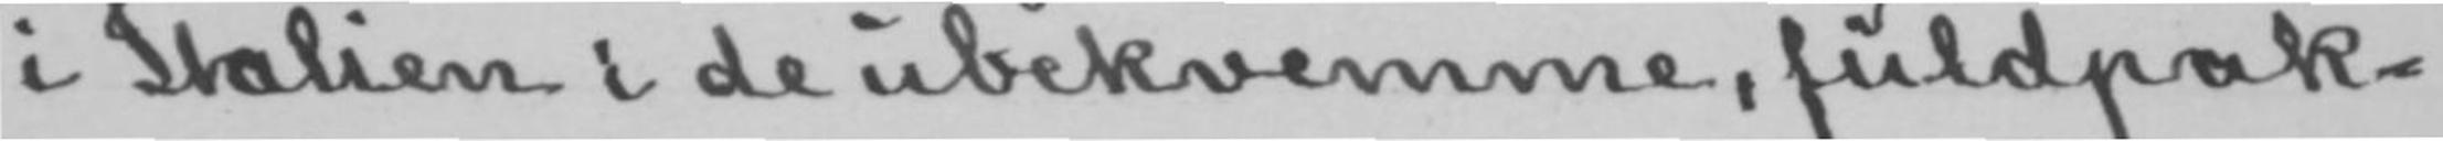i Italien i de ubekvemme, fuldpak-
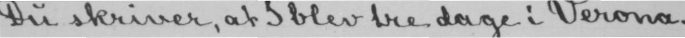Du skriver, at I blev tre dage i Verona.
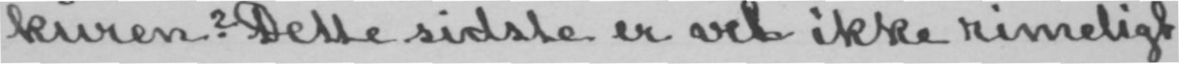kuren? Dette sidste er vel ikke rimeligt
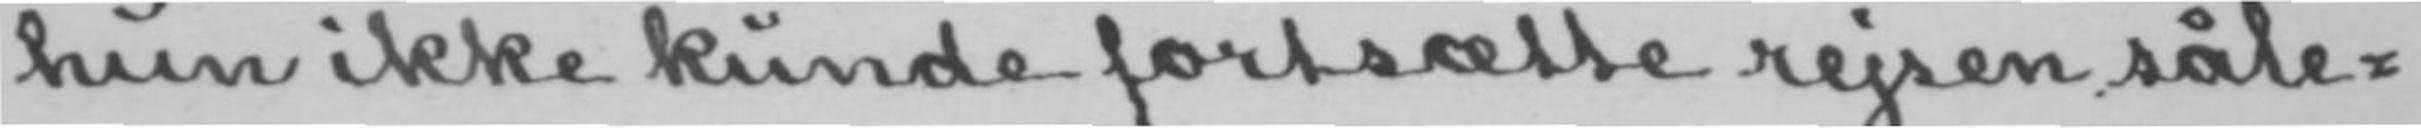hun ikke kunde fortsætte rejsen såle-
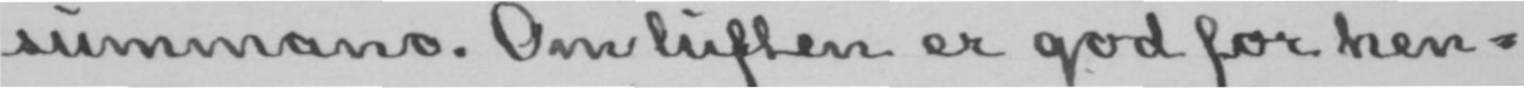summano. Om luften er god for hen-
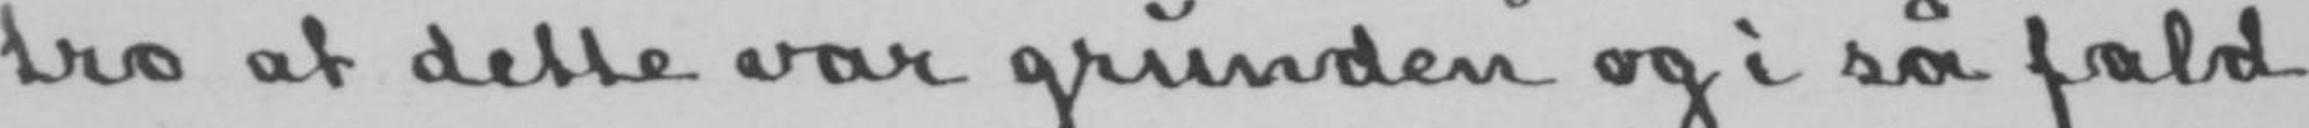tro at dette var grunden og i så fald
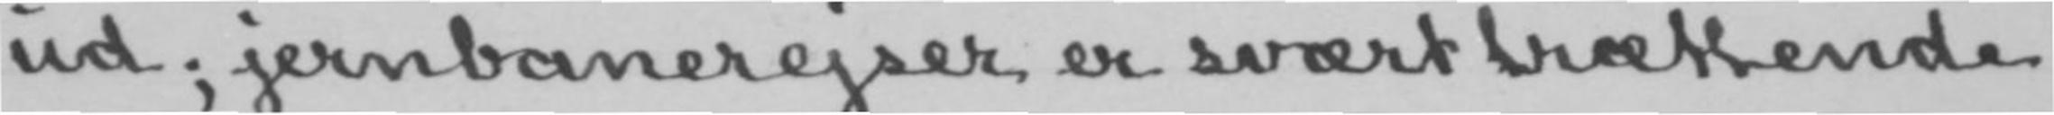ud; jernbanerejser er svært trættende
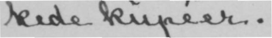kede kupéer.
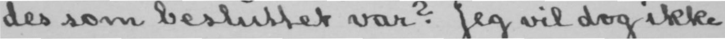des som besluttet var? Jeg vil dog ikke
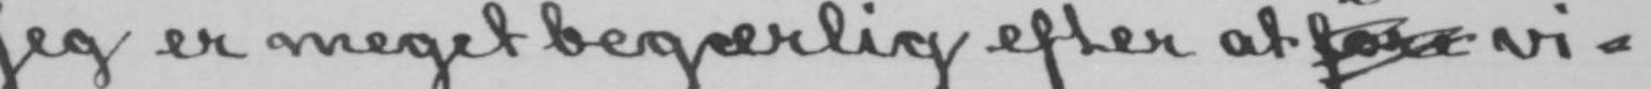Jeg er meget begærlig efter at føre vi-
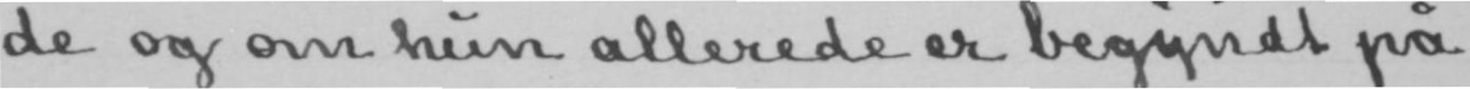de og om hun allerede er begyndt på
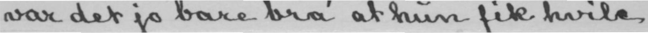var det jo bare bra' at hun fik hvile
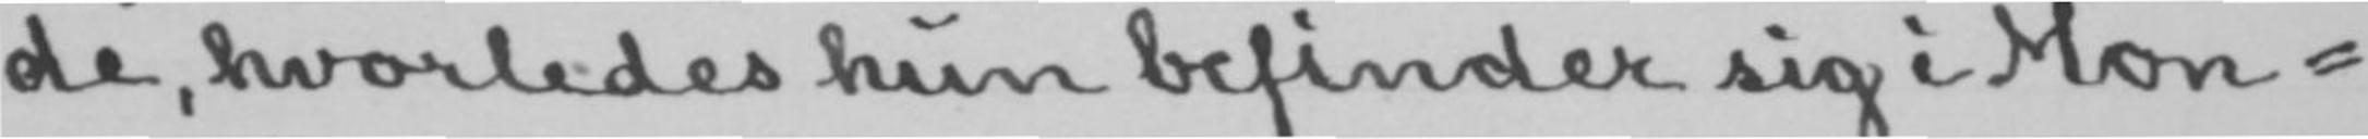de, hvorledes hun befinder sig i Mon-
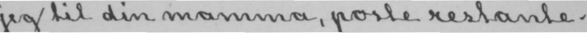jeg til din mamma, poste restante.
